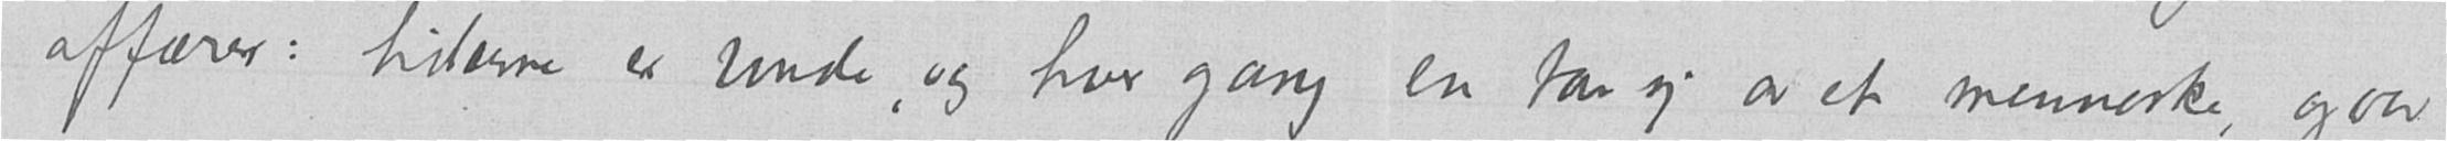affærer: tiderne er vonde, og hver gang en tar sig av et menneske, gaar
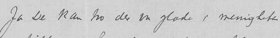Ja De kan tro der var glæde i menigheten
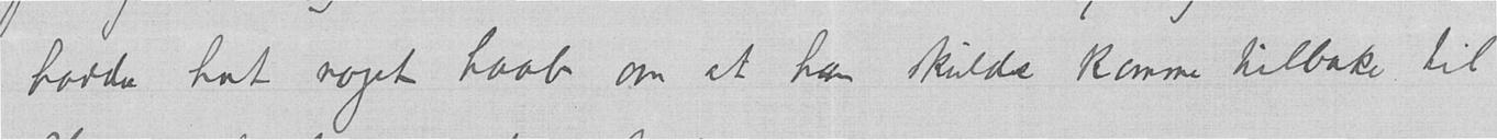hadde hat noget haab om at han skulde komme tilbake til
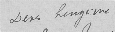Deres hengivne
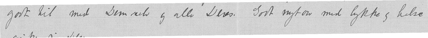godt til med Dem selv og alle Deres. Godt nytaar med lykke og helse
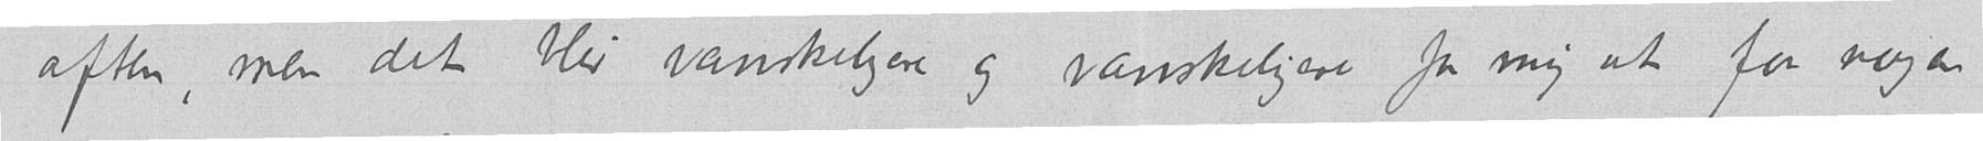aften, men det blir vanskeligere og vanskeligere for mig at faa nogen
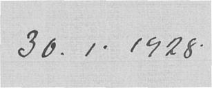30.1.1928.
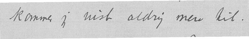kommer jeg vist aldrig mere til.
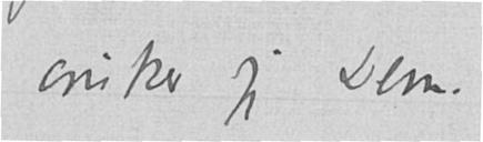ønsker jeg Dem.
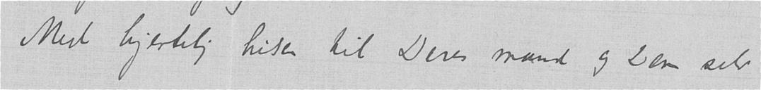Med hjertelig hilsen til Deres mand og Dem selv
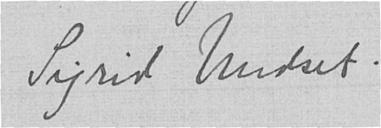Sigrid Undset.
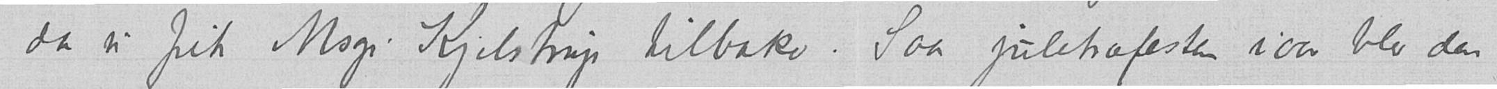da vi fik Msgr. Kjelstrup tilbake. Saa juletræfesten iaar blev den
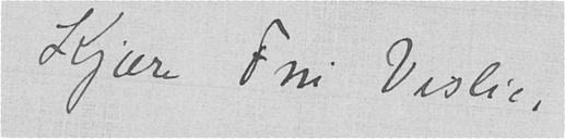Kjære Fru Vislie,
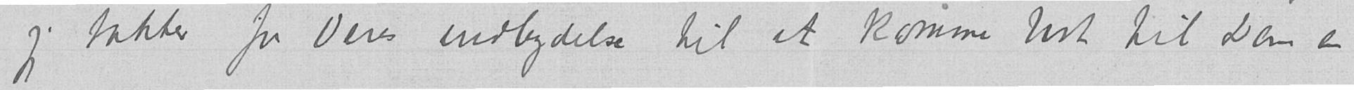jeg takker for Deres indbydelse til at komme bort til Dem en
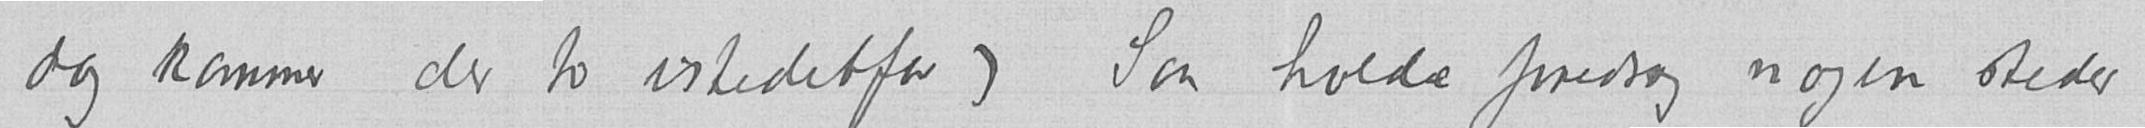dag kommer der to istedetfor) Saa holde foredrag nogen steder
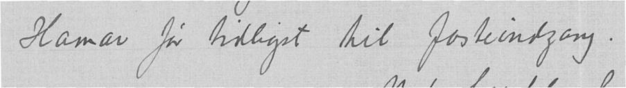Hamar før tidligst til fasteindgang.
